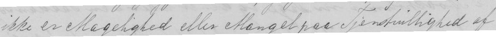ikke er Magelighed eller Mangel paa Tjenstvillighed af
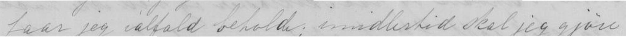faar jeg ialfald beholde; imidlestid skal jeg gjøre
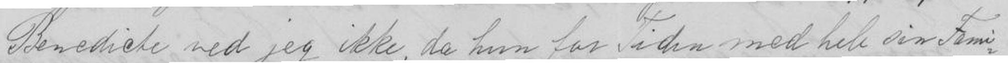Benedicte ved jeg ikke, da hun for Tiden med hele Din Fami-
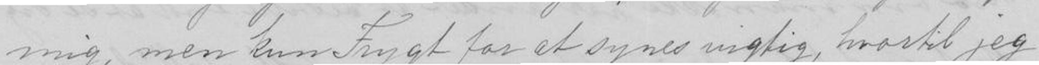mig, men kun Frygt for at synes vigtig, hvortil jeg
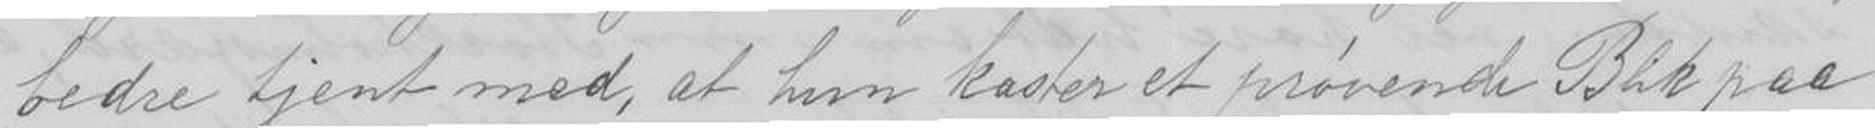bedre tjent med, at hun kaster et prøvende Blik paa
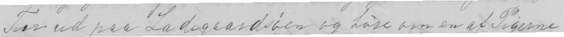Tur ud paa Laadegaardsien og høre om en af Pigerne
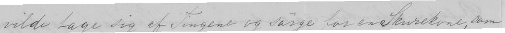vilde tage sig af Tingene og sørge for en Skurekone, som
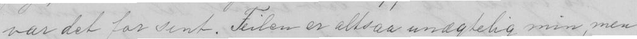var det for sent. Feilen er altsaa unægtelig min, men
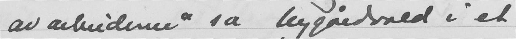av arbeiderne" sa Nygårdsvold i et
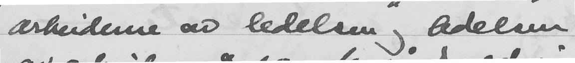arbeiderne av ledelsen og ledelsen
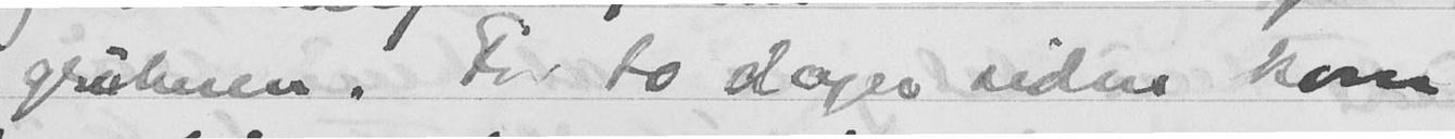gruben. For to dager siden kom
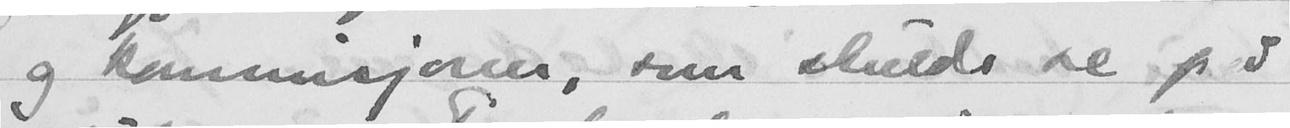og kommisjoner, som skulde se på
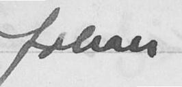Johan
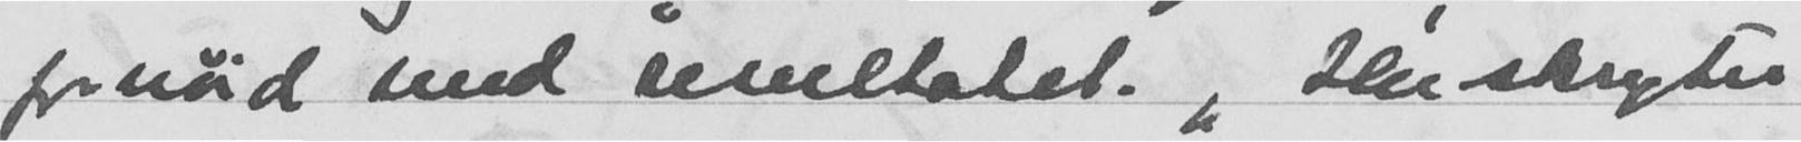fornøid med resultatet. "Her skryter
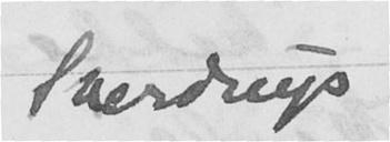Sverdrups
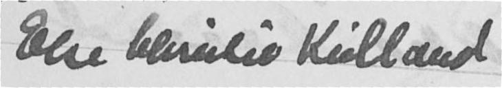Else Christie Kielland
